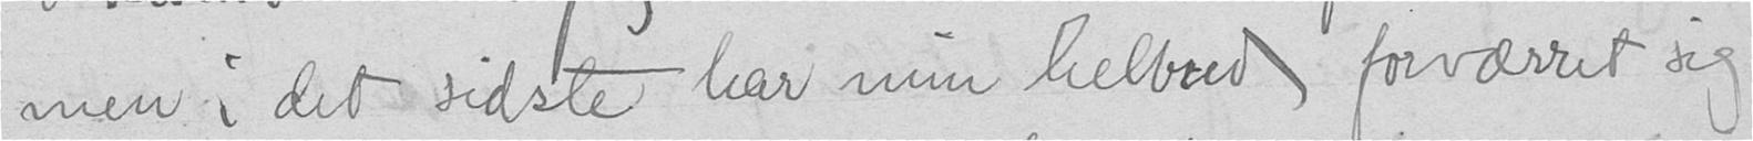men i det sidste har min helbred forværret sig
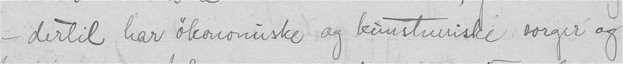- dertil har økonomiske og kunstneriske sorger og
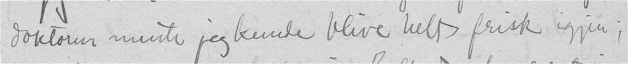doktoren mente jeg kunde blive helt frisk igjen;
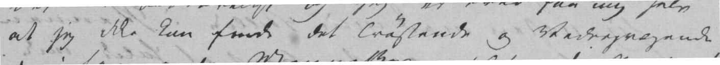at jeg ikke kan finde det Trøstende og Vederqvægende
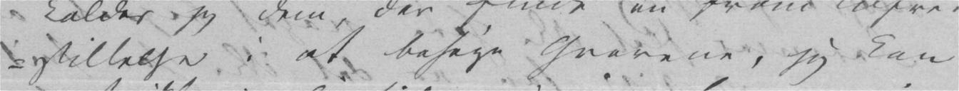stillelse i at besøge Gravene, jeg kan
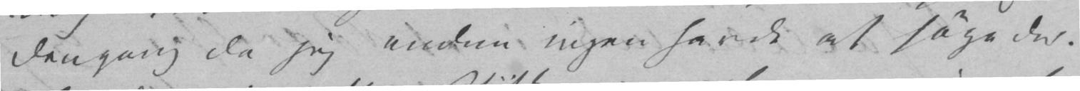dengang da jeg endnu ingen havde at søge der.
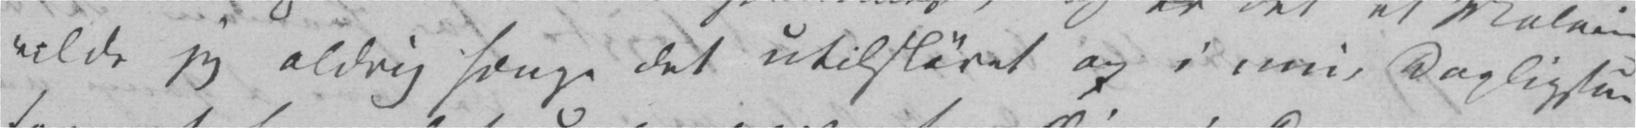vilde jeg aldrig hænge det utilsløret op i min Dagligstue
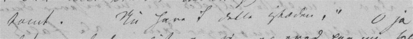tomt. Nu have I dette istæden,» O ja
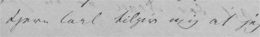Kjere Carl tilgiv mig at jeg
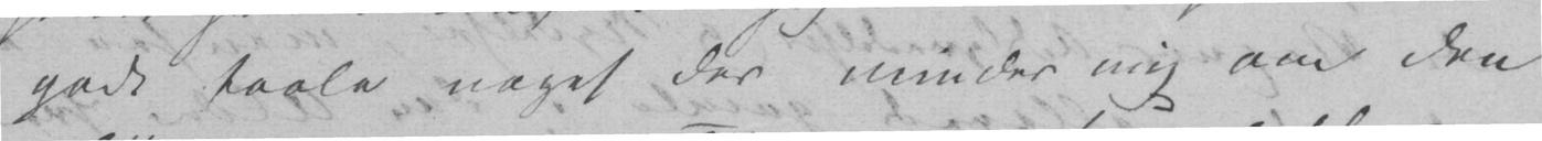godt taale noget der minder mig om den
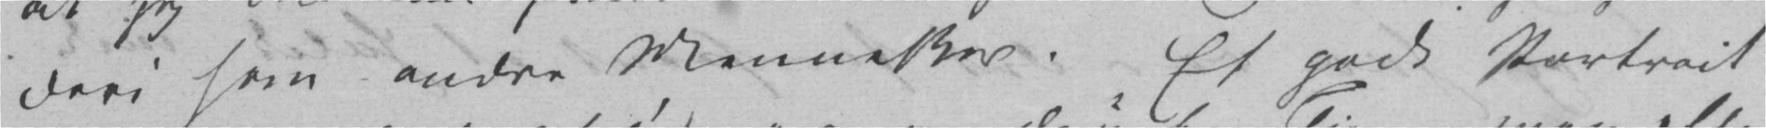deri som andre Mennesker. Et godt Portrait
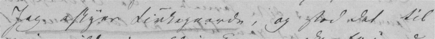Jeg afskyer Kirkegaarde, og stod det til
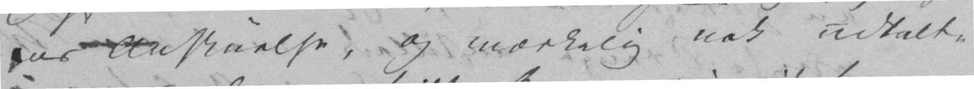ens Anskuelse, og mærkelig nok udtalte
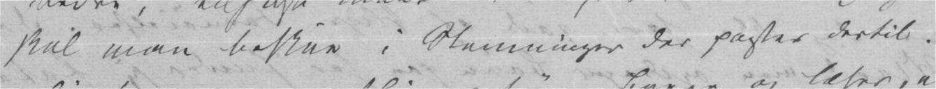skal man beskue i Stemninger der passer dertil.
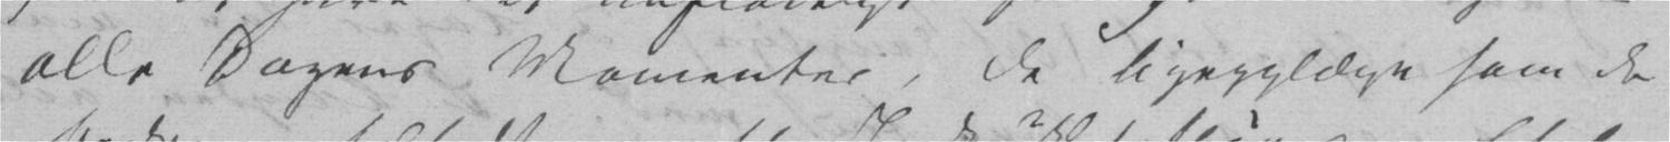alle Dagens Momenter, de ligegyldige som de
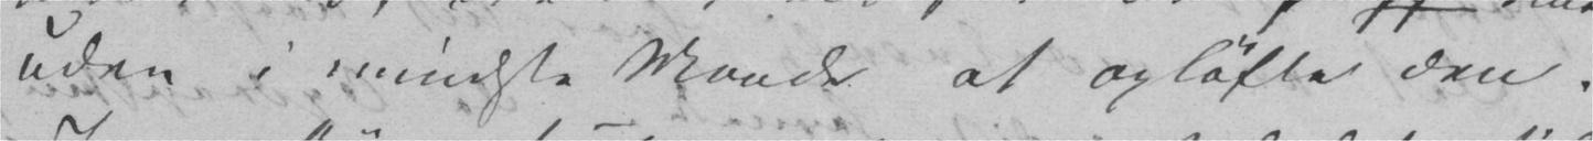uden i mindste Maade at opløfte den.
